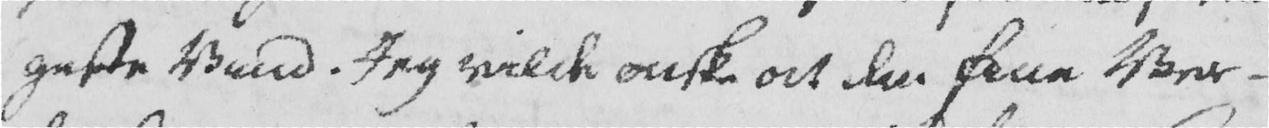geste Vind. Jeg vilde ønske at den fine Ver-
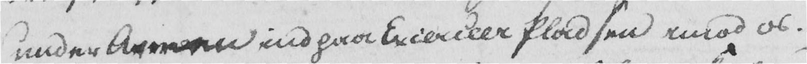under Armen ind paa Excerceer Pladsen imod os.
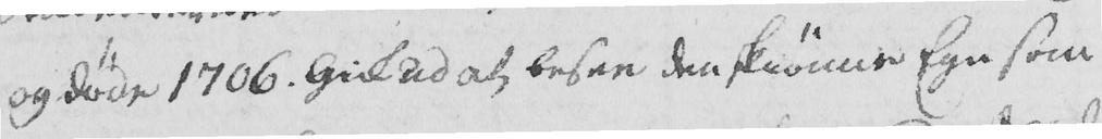og døde 1706. Gik ud at besee den skiønne Egn som
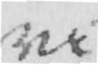vi
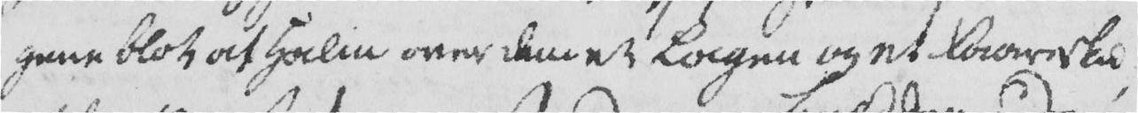gene blot af Halm over dem et Lagen og et Faareskind,
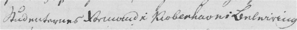Studenternes Formand i Kiøbenhavns Beleiring
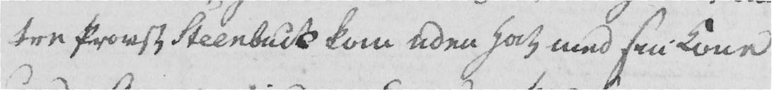tre Provst Steenbuck kom uden Hat med sin Kone
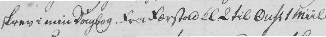skrev i min Dagbog. Fra Færstad kl. 2 til Oust 1 Miil
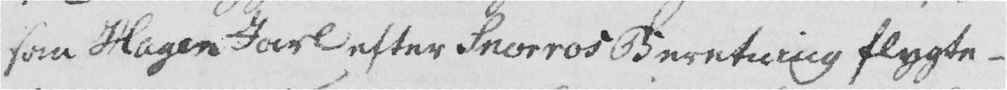som Hagen Jarl efter Snorros Beretning flygte-
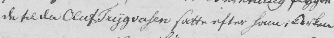de til da Oluf Trygvasen satte efter ham; Kirken
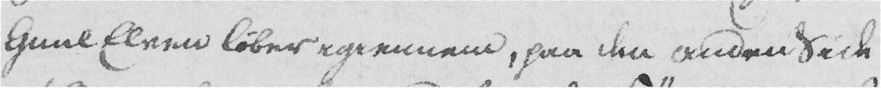Guul Elven løber igiennem, paa den anden side
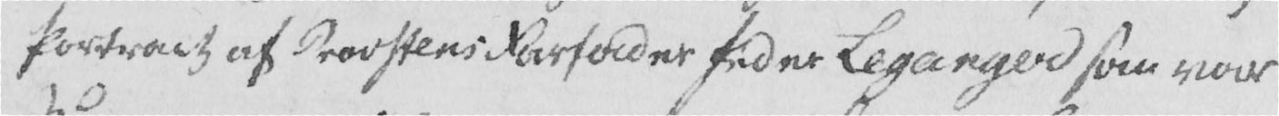Portrait af Provstens Forfader Peder Leganger, som var
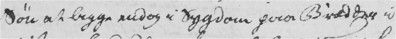Søn at ligge endog i Sygdom paa Brædder i
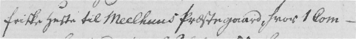friske Heste til Meelhuus Præstegaard, hvor 1 Com-
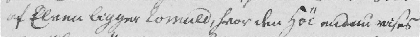af Elven ligger Romuld, hvor den Høi endnu vises
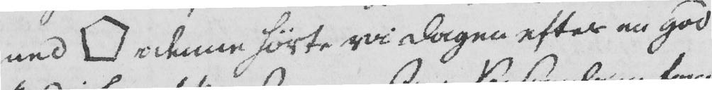ned  i denne hørte vi dagen efter en god
